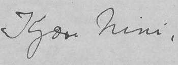Kjære Nini,
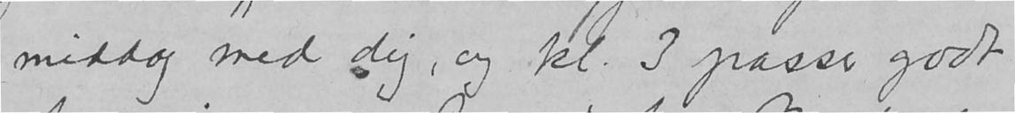middag med dig, og kl. 3 passer godt
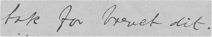tak for brevet dit.
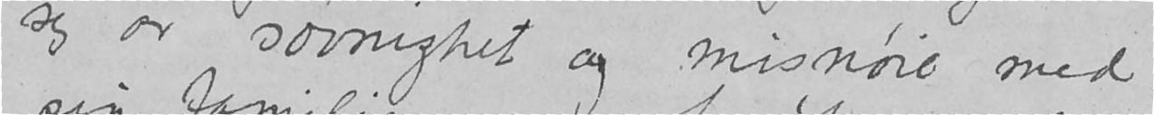sig av søvnighet og misnøie med
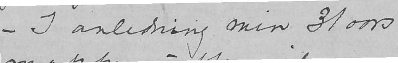– I anledning min 31 aars
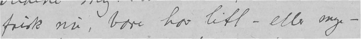frisk nu bare har litt – eller mye –
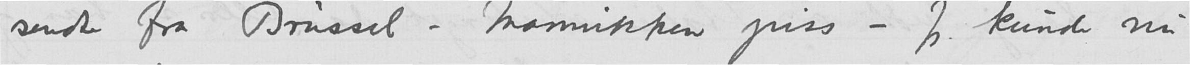sendte fra Brussel – Mannikken piss – Jeg kunde nu
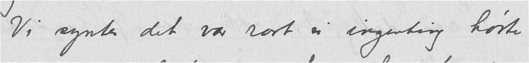Vi syntes det var rart vi ingenting hørte
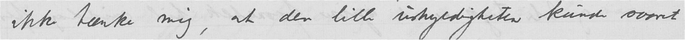ikke tænke mig, at den lille uskyldigheten kunde saaret
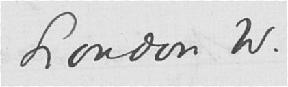London W.
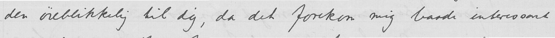den øieblikkelig til dig, da det forekom mig baade interessant
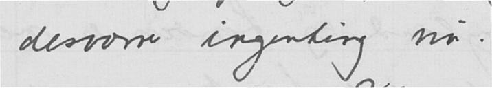desværre ingenting nu.
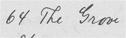64 The Grove
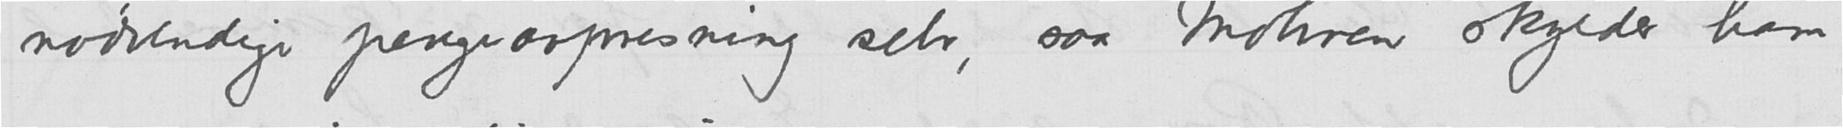nødvendige pengeavpresning selv, saa Mohren skylder ham
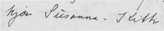kjære Susanna - Kitti,
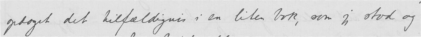opdaget det tilfældigvis i en liten bok, som jeg stod og
